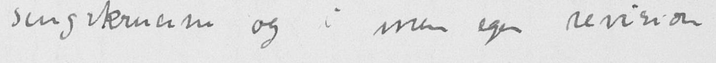singskruerne og i min egen revision
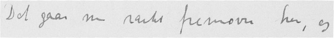Det gaar nu raskt fremover her, og
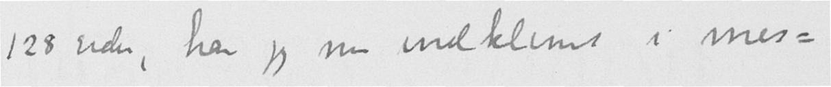128 sider, har jeg nu indklemt i mes-
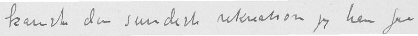kanske den sundeste rekreation jeg kan faa
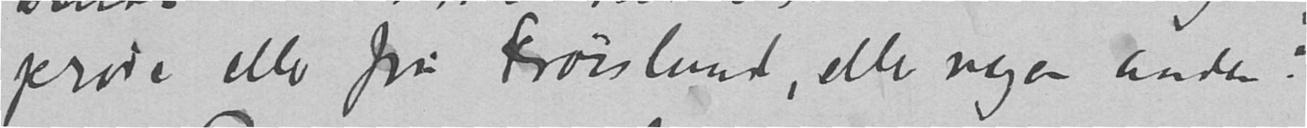prøve eller fru Frøisland, eller nogen anden?
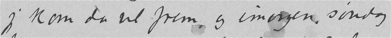jeg kom da vel frem, og imorgen, søndag
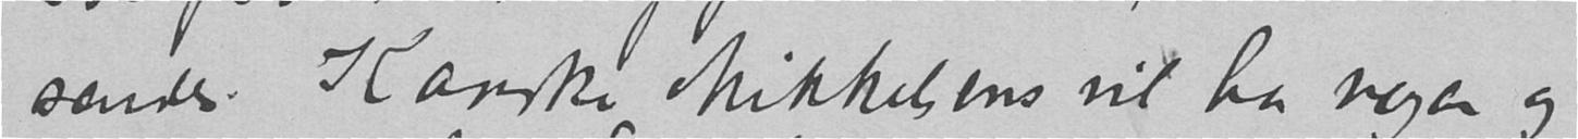sendes. Kanske Mikkelsens vil ha nogen og
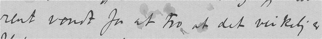rent vondt for at tro at det virkelig er
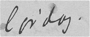lørdag.
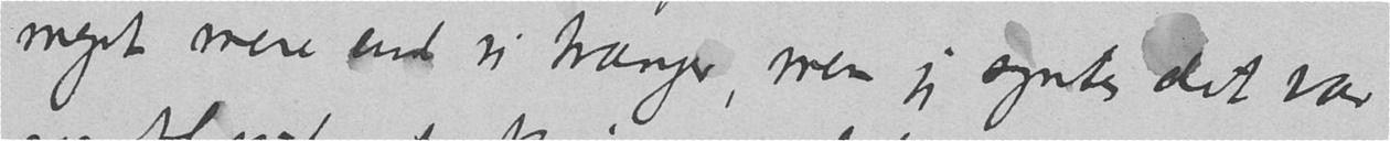meget mere end vi trænger, men jeg syntes det var
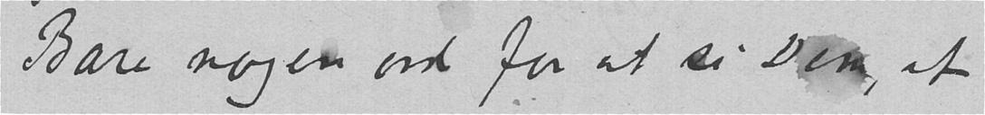Bare nogen ord for at si Dem, at
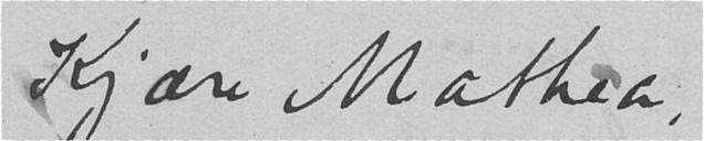Kjære Mathea,
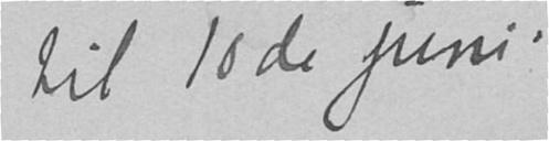til 10de juni.
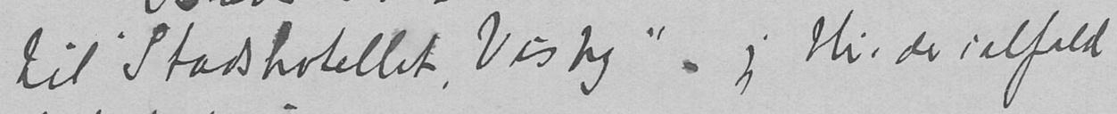til "Stadshotellet, Visby" – jeg blir der ialfald
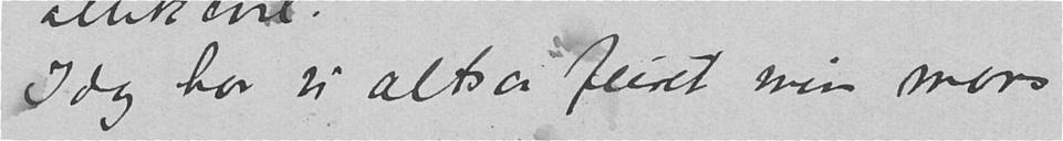Idag har vi altsaa feiret min mors
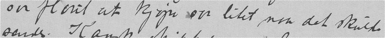saa flaut at kjøpe saa litet, naar det skulde
